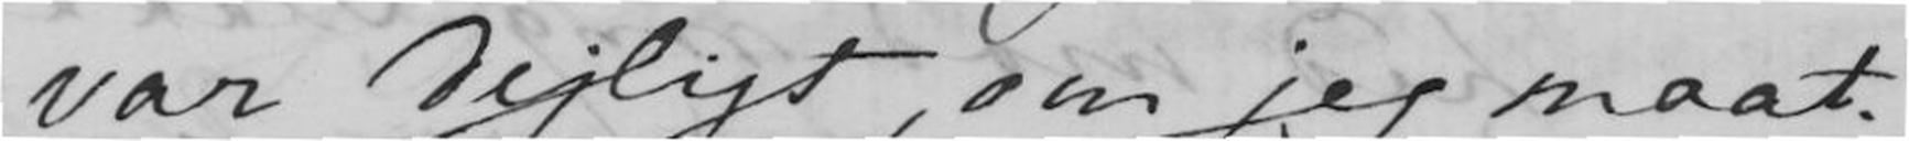var dejligt, om jeg maat-
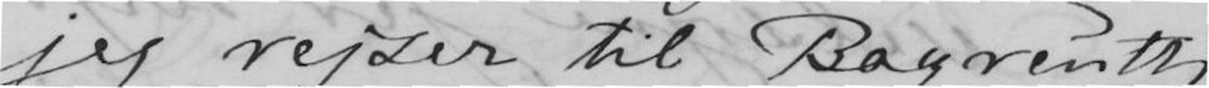jeg rejser til Bayreuth,
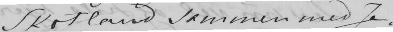Skotland sammen med Ja-
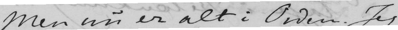Men nu er alt i Orden. Jeg
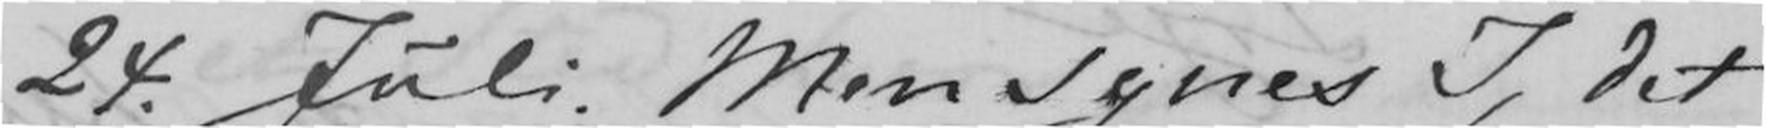24 Juli. Men synes I, det
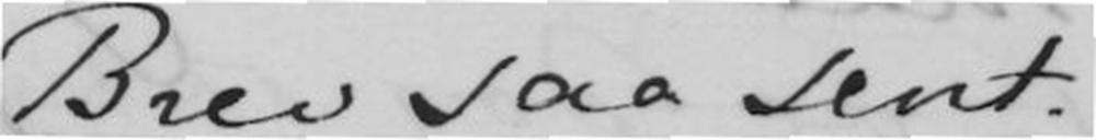Brev saa sent.
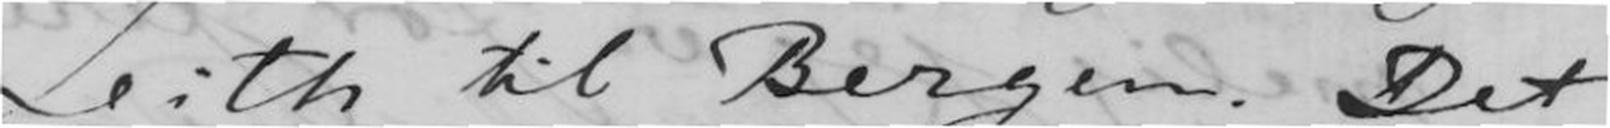Leith til Bergen. Det
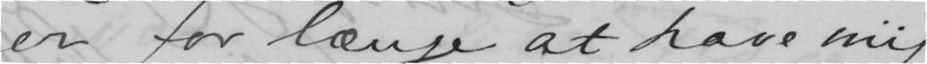er for længe at have mig
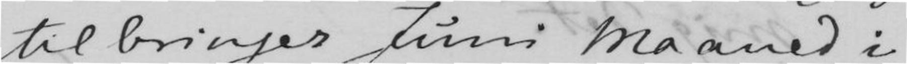tilbringer Juni Maaned i
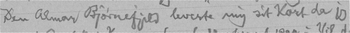Den Almar Bjørnefjeld leverte mig sit Kort da jeg
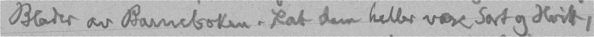Blader av Barneboken. Lat dem heller være Sort og Hvitt,
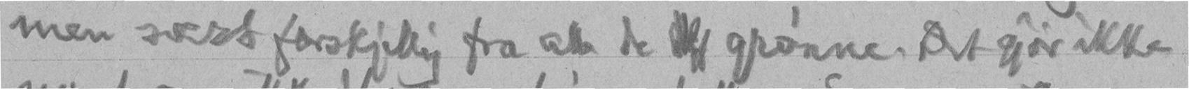men svert forskjellig fra alle de  grønne. Det gjør ikke
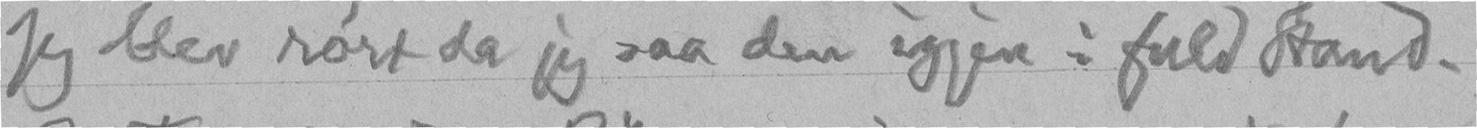jeg blev rørt da jeg saa den igjen i fuld Stand.
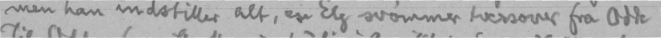men han indstiller alt, en Elg svømmer tversover fra Odde
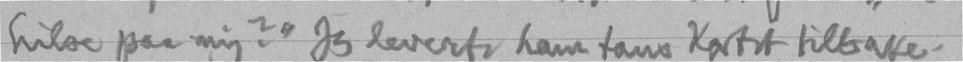hilse paa mig?" Jeg leverte ham taus Kortet tilbake.
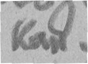kan.
